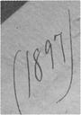(1897)
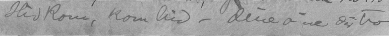Hid kom, kom hid - dine øine du tro
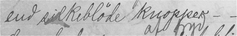end silkebløde knopper - -
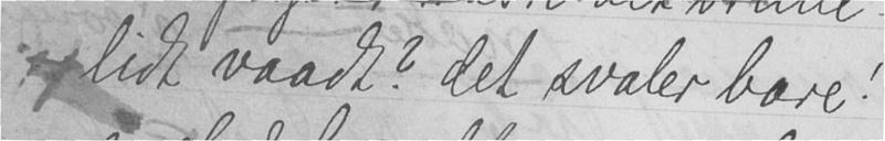lidt vaadt? det svaler bare!
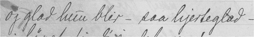og glad hun blir - saa hjerteglad -
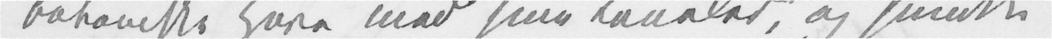botaniske Have med sine Kanaler, og smukke
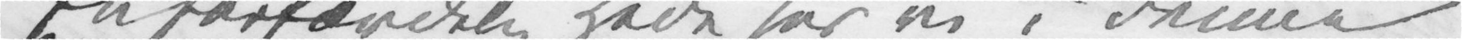En forfærdelig Hede har vi i denne
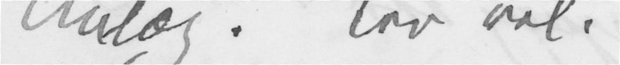Anlæg. Lev vel.
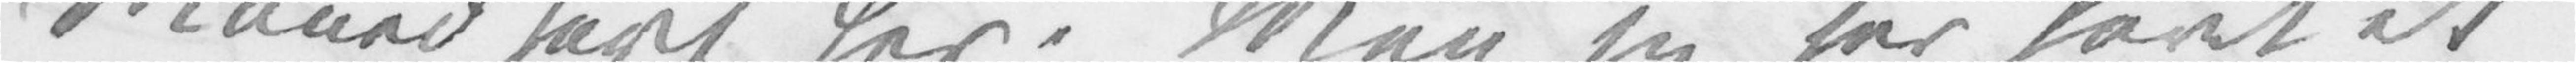Måned havt her. Men jeg har havt et
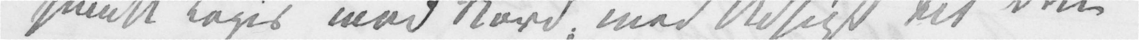smukt Logis mod Nord, med Udsigt til den
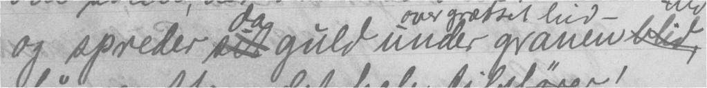og spreder da guld under granen hid
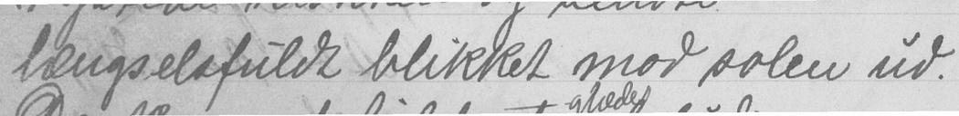længselsfuldt blikket mod solen ud.
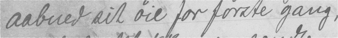aabned sit øie for første gang,
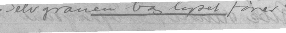Selv granen og lyset fører!
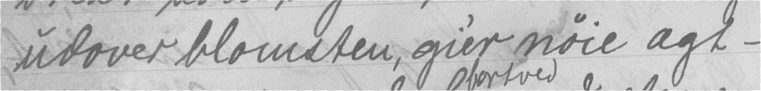udover blomsten, gi'er nøie agt -
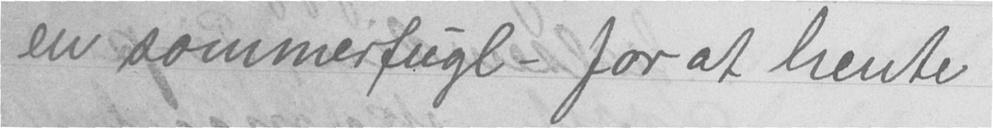en sommerfugl - for at hente
